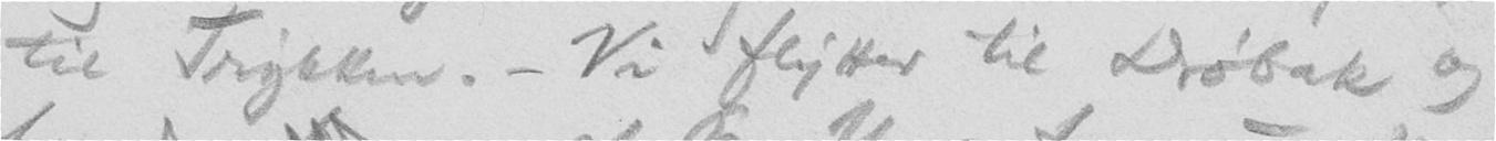til Trykken. - Vi flytter til Drøbak og
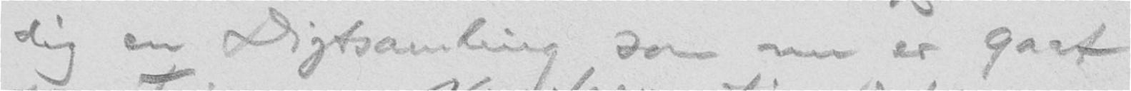dig en Digtsamling som nu er gaat
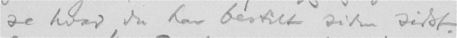se hvad du har bestilt siden sidst.
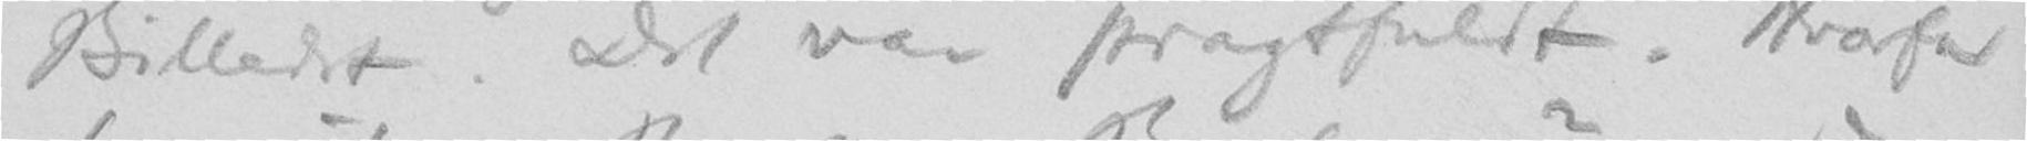Billedet. Det var pragtfuldt. Hvorfor
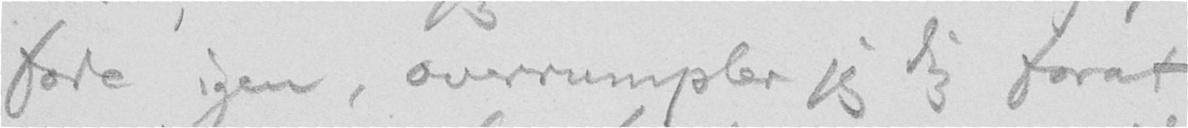fode igen, overrumpler jeg dig forat
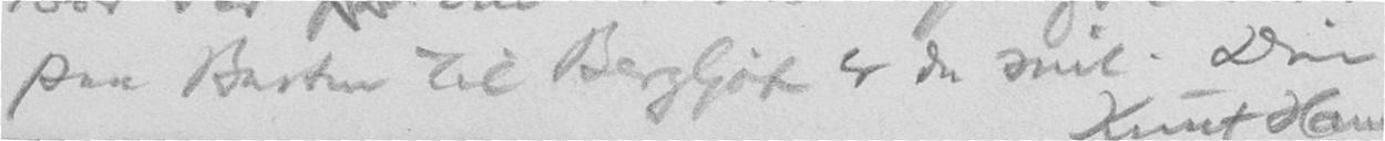paa Busten til Bergljot er du snil. Din
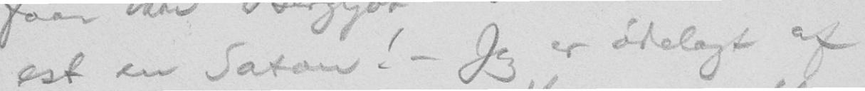est en Satan! - Jeg er ødelagt af
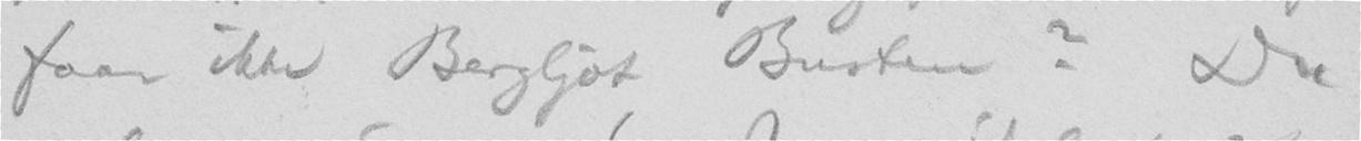faar ikke Bergljot Busten? Du
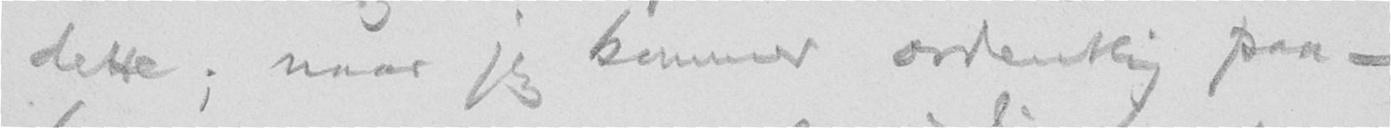dette; naar jeg kommer ordentlig paa-
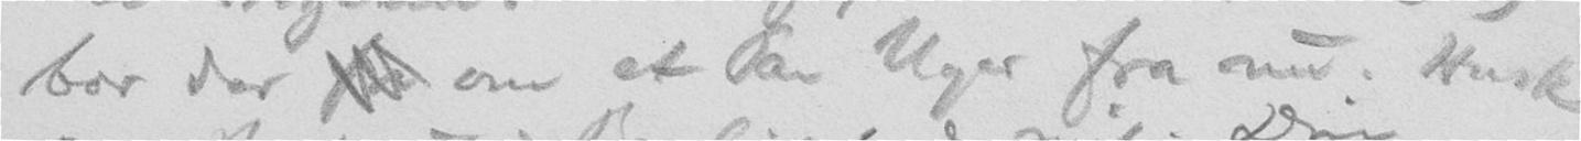bor der fra om et Par Uger fra nu. Husk
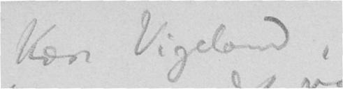Kære Vigeland,
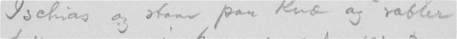Ischias og staar paa Knæ og rabler
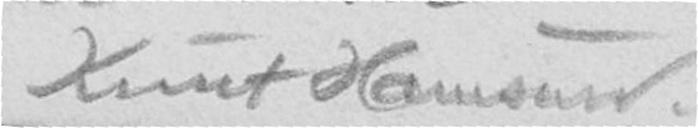Knut Hamsun.
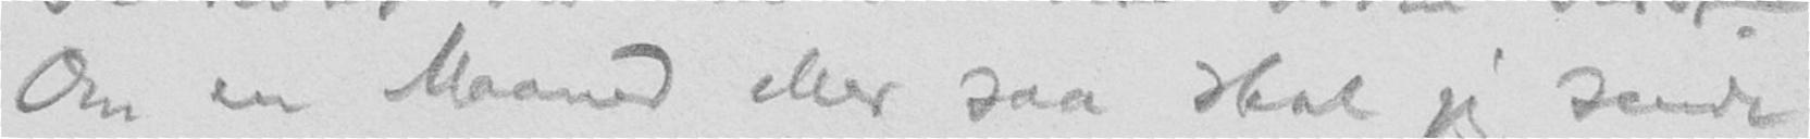Om en Maaned eller saa skal jeg sende
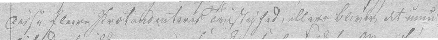disse flere Prætendenteres Tvistighed, ellers bliver det umu-
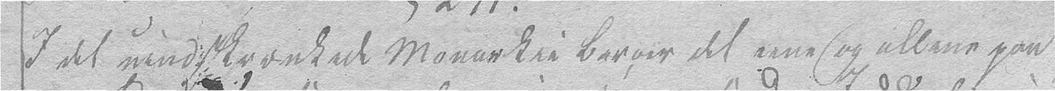I det uindskrænkede Monarkie beroer det eene og allene paa
